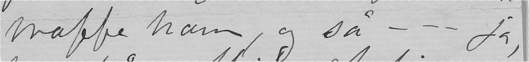træffe ham, og så - - - Ja,
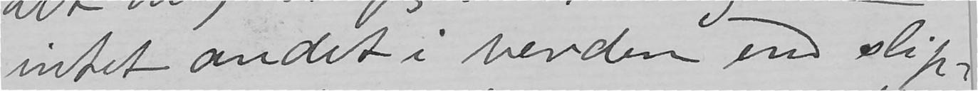intet andet i verden end slip-
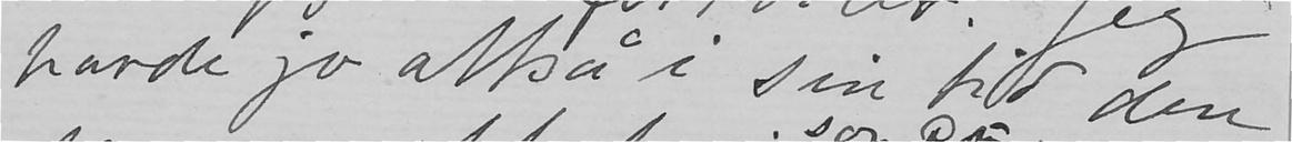havde jo altså i sin tid den
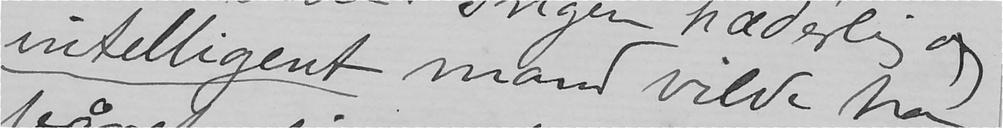intelligent mand vilde ha
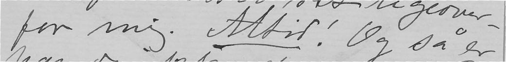for mig. Altid! Og så er
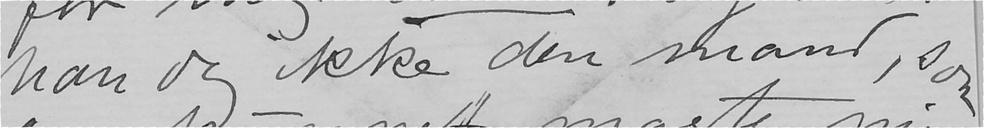han dog ikke den mand, som
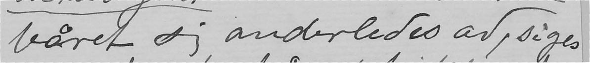båret sig anderledes ad, siges
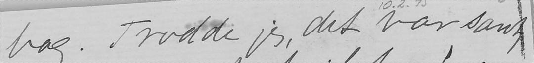bog. Trodde jeg, det var sant,
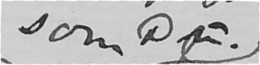som Du;
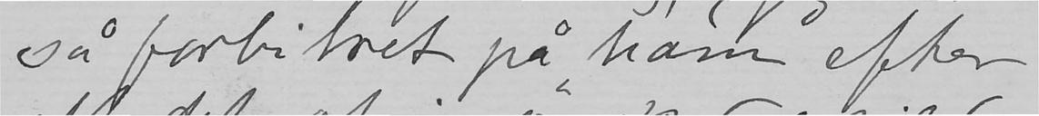så forbitret på ham efter
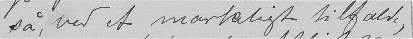så, ved et markeligt tilfælde,
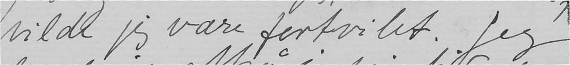vilde jeg være fortvilet. Jeg
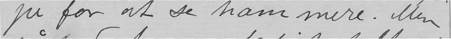pe for at se ham mere. Men
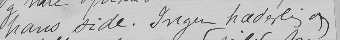hans side. Ingen hæderlig og
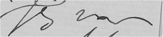jeg var
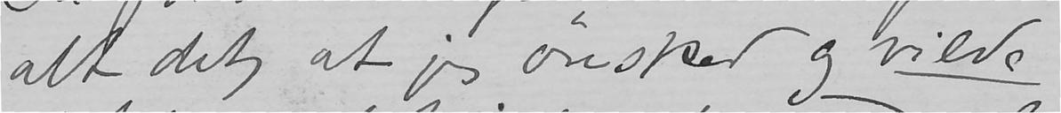alt det at jeg ønsked og vilde
